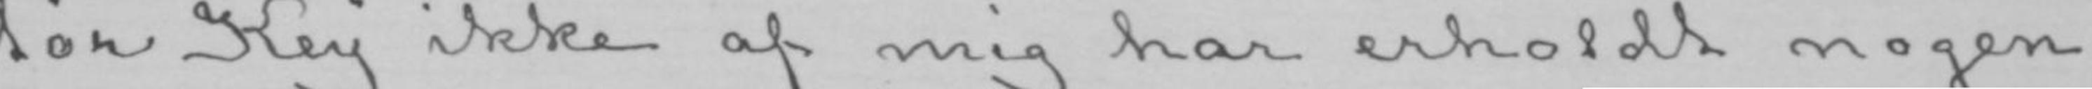tør Key ikke af mig har erholdt nogen
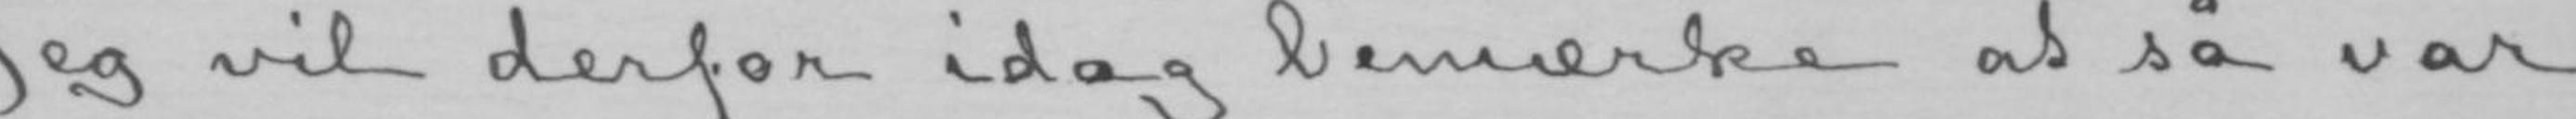Jeg vil derfor idag bemærke at så var
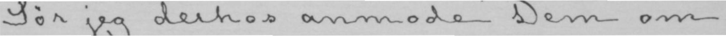STør jeg derhos anmode Dem om,
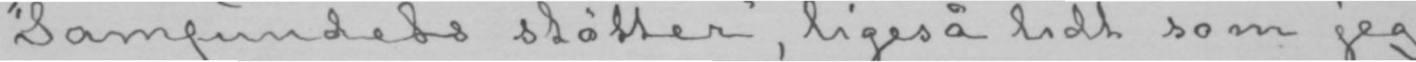«Samfundets støtter», ligeså lidt som jeg
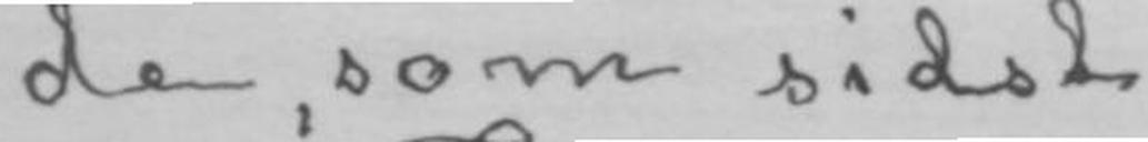de, som sidst.
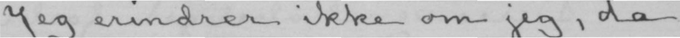Jeg erindrer ikke om jeg, da
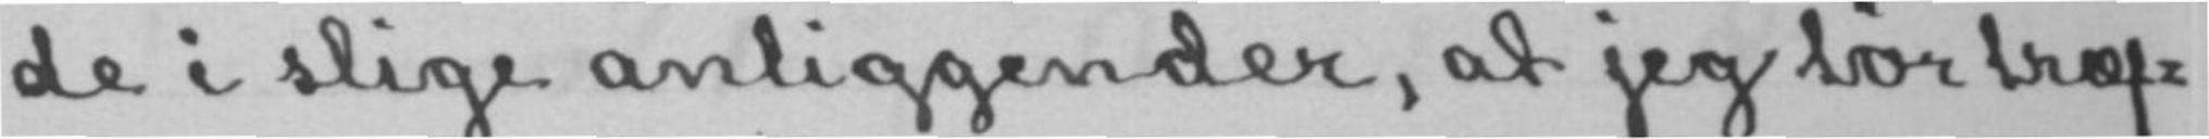de i slige anliggender, at jeg tør træf-
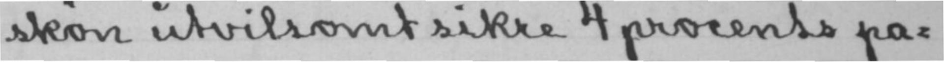skøn utvilsomt sikre 4 procents pa-
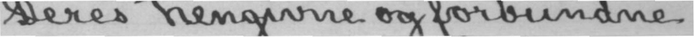Deres hengivne og forbundne
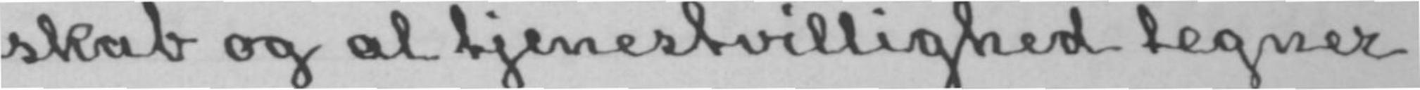skab og al tjenestvillighed tegner
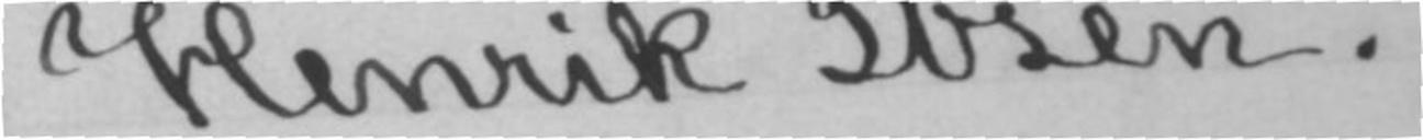Henrik Ibsen.
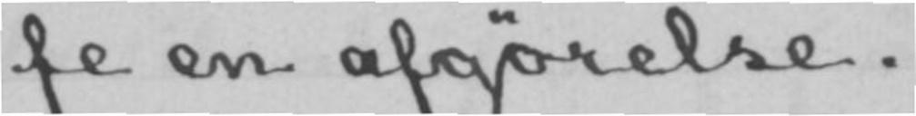fe en afgørelse.
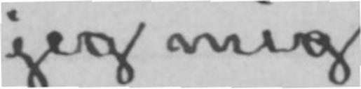jeg mig
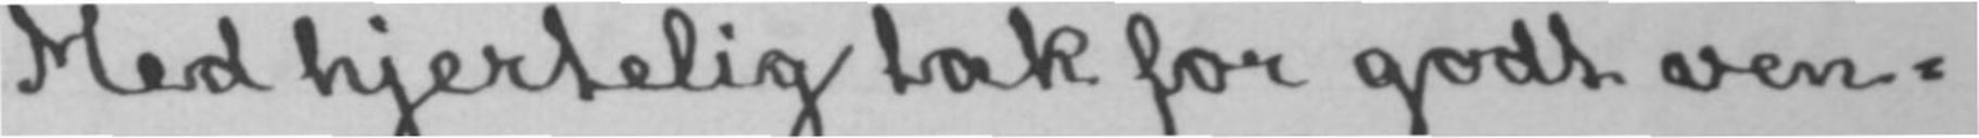Med hjertelig tak for godt ven-
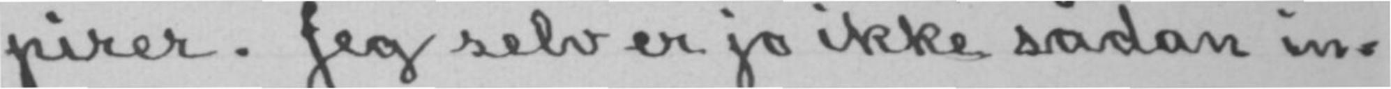pirer. Jeg selv er jo ikke sådan in-
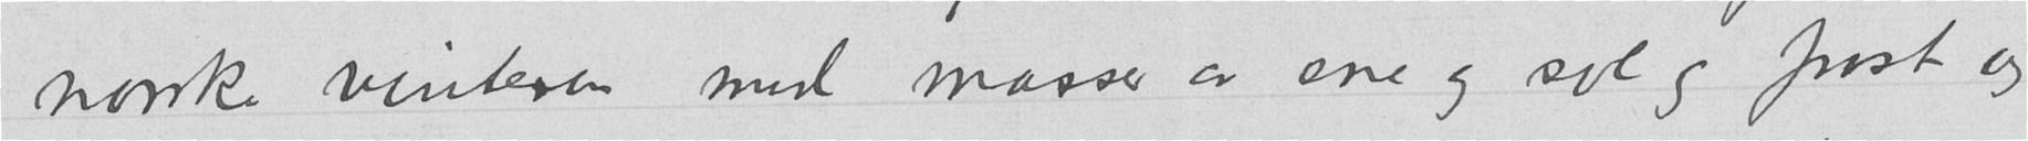norske vinteren med masser av sne og sol og frost og
